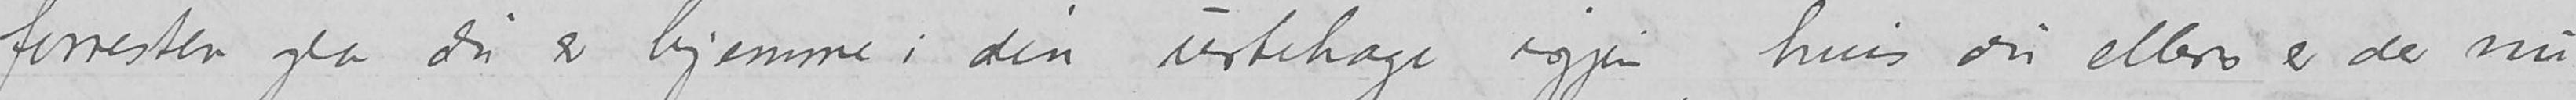forresten gla du er hjemme i din urtehage igjen, hvis du ellers er der nu
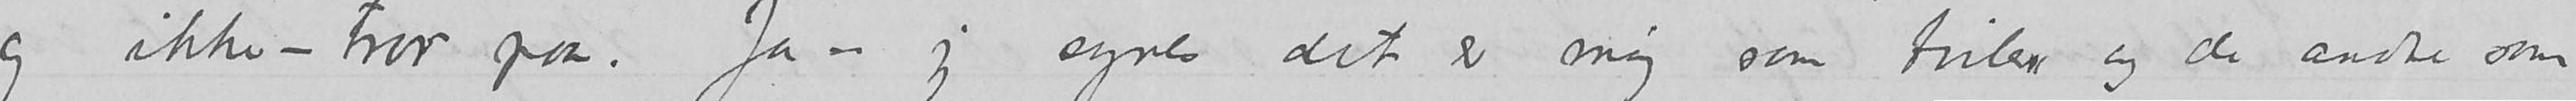og ikke – tror paa.  Ja – jeg synes det er mig som tviler og de andre som
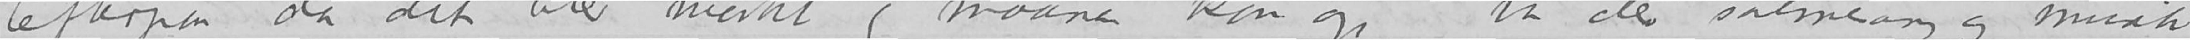.  Efterpaa, da det blev mørkt og maanen kom op, var der salmesang og musik
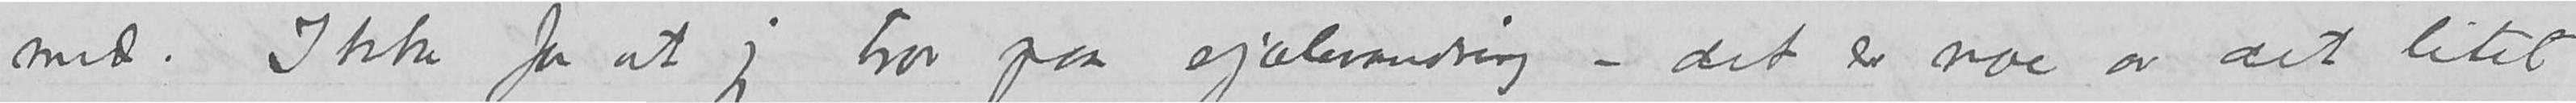med.  Ikke for at jeg tror paa sjælevandring – det er noe av det litet
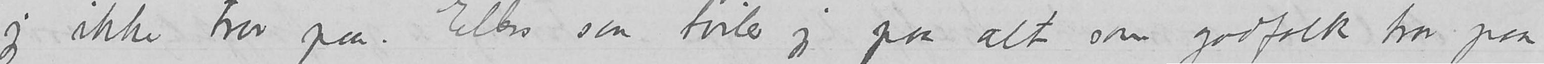jeg ikke tror paa.  Ellers saa tviler jeg paa alt som godtfolk tror paa
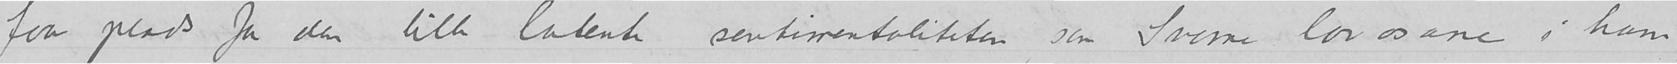faar plads for den lille latente sentimentaliteten som Snorre lar os ane i ham,
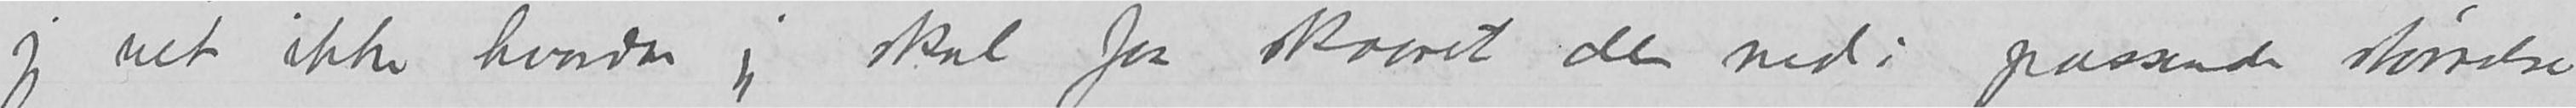jeg vet ikke hvordan jeg skal faa skaaret den ned i passende størrelse,
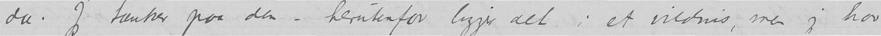da.  Jeg tænker paa den – herutenfor ligger det i et vildnis, men jeg har
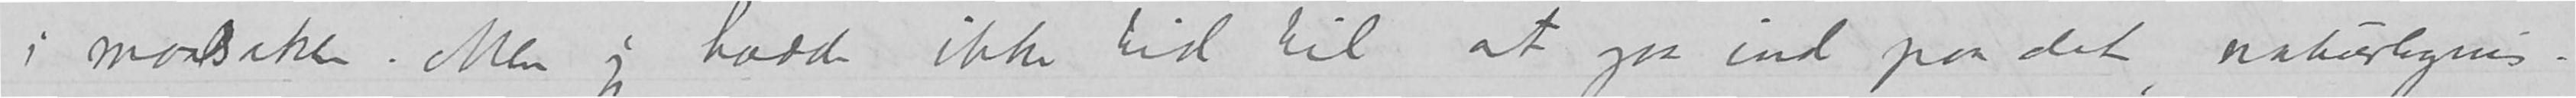i mordsaken/mordakten.  Men jeg hadde ikke tid til at gaa ind paa det, naturligvis
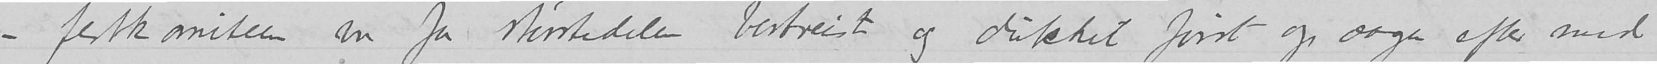– festkomiteen var for størstedelen bortreist og dukket først op dagen efter med
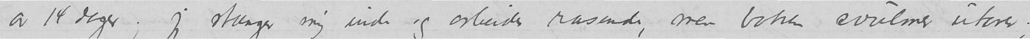av 14 dager;  jeg stænger mig inde og arbeider rasende, men boken svulmer utover;
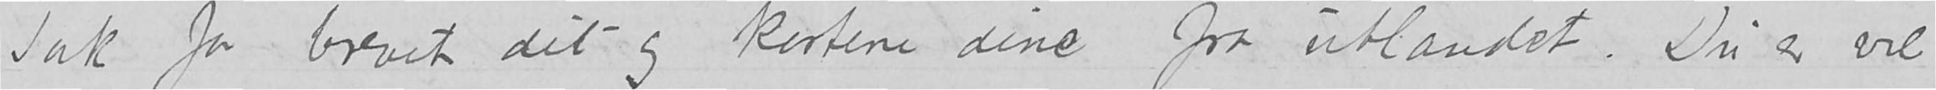Tak for brevet dit og kortene dine fra utlandet.  Du er vel
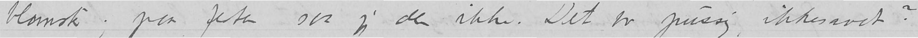blomster; paa festen saa jeg dem ikke.  Det var pussig, ikkesandt?
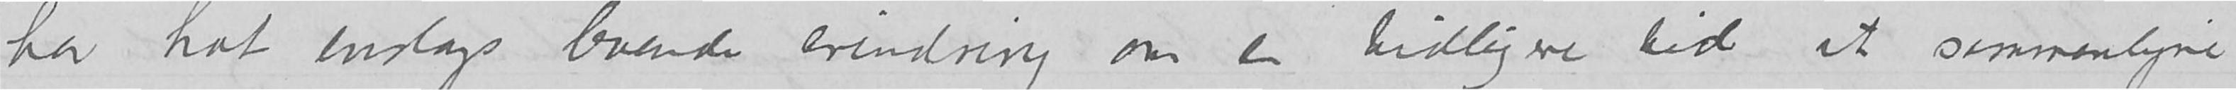har hat enslags levende erindring om en tidligere tid at sammenligne
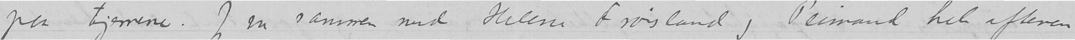paa tjernene.  Jeg var sammen med Helene Frøisland og Peimand hele aftenen
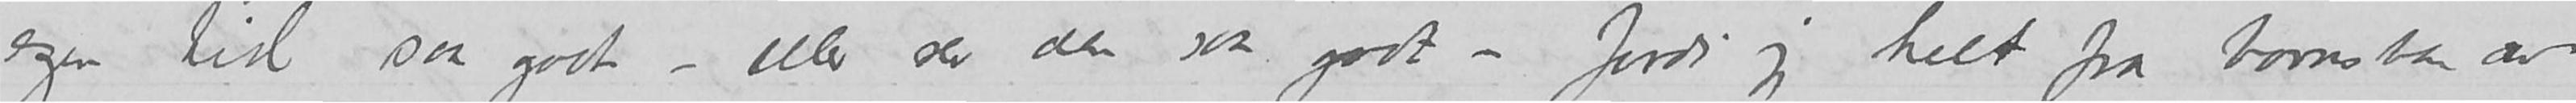egen tid saa godt – eller ser den saa godt – fordi jeg helt fra barnsben av
